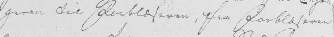geren til Forblæseren, fra Forblæseren
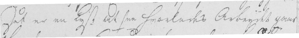Det er en Lyst at see hvorledes Arbeydet gaar
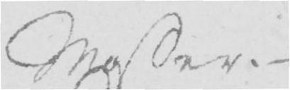Masser. -
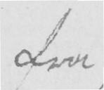fra
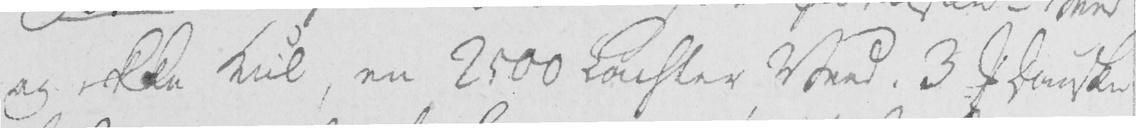og ikke Kul, en 2500 Lansler Veed. 3 D Danske
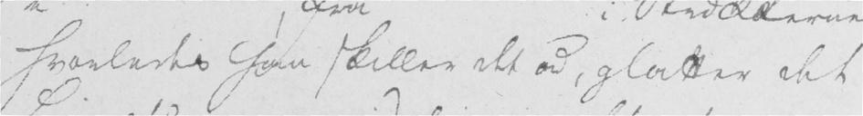hvorledes han skiller det ud, glatter det
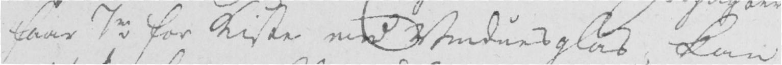faar 7 r for Liste med Vinduesglas, kan
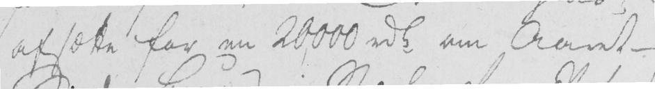afsætte for en 20,000 rde om Aaret -
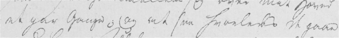et par Gange; og at see hvorledes de gaar
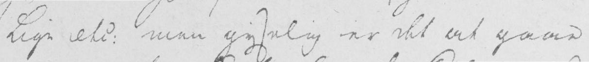Ligt etc. men gyselig er det at gaae
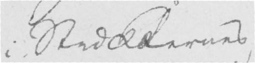i Stedkeovnen,
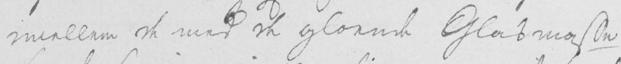imellem de med de gloende Glasmasse
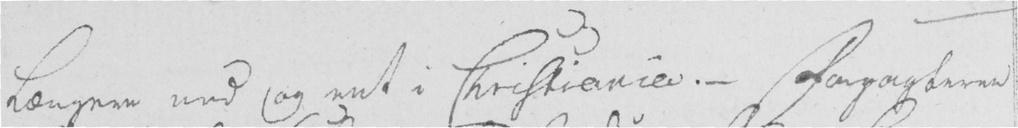længere ned og ret i Christiania. - Forpagteren
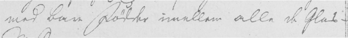med bare Fødder imellem alle de Glas-
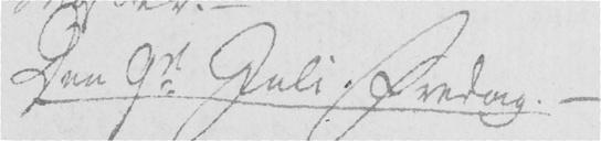Den 9de Juli Fredag. -
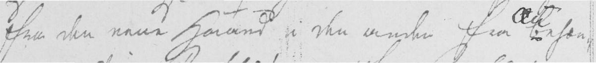fra den eene Haand i den anden fra behæn-
